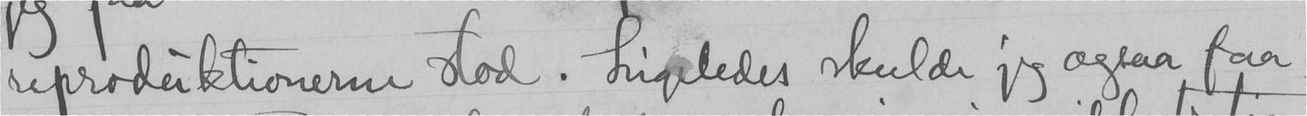reproduktionerne stod. Ligeledes skulde jeg ogsaa faa
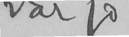var jo
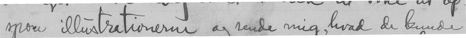spore illustrationerne og sende mig, hvad de kunde
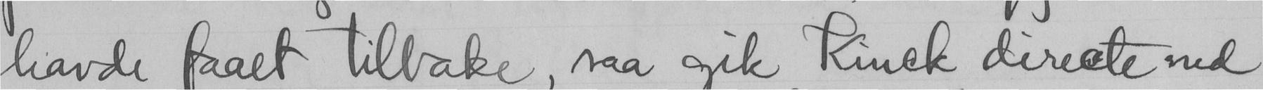havde faaet tilbake saa gik Kinck directe ned
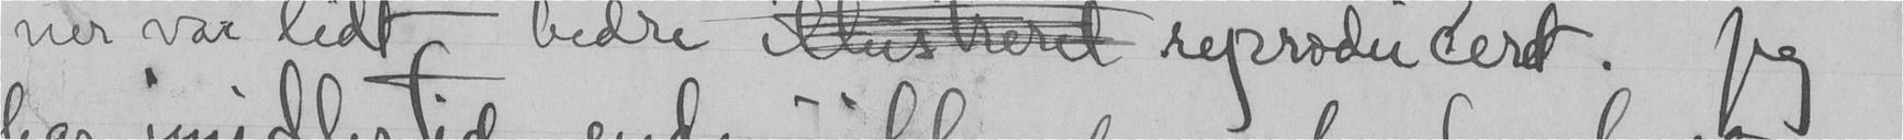ner var lidt bedre  reproducert. Jeg
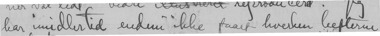har imidlertid endnu ikke faaet hverken hefterne
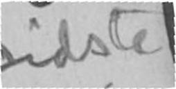sidste
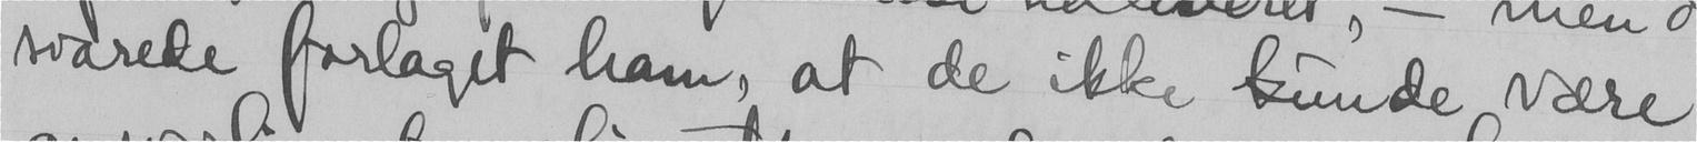svarede forlaget ham, at de ikke kunde være
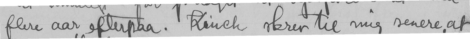flere aar efterpaa. Kinck skrev til mig senere, at
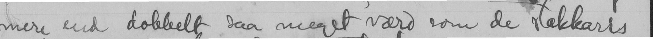mere end dobbelt saa meget værd som de stakkars
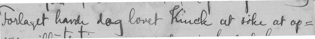Forlaget havde dog lovet Kinck at søke at op-
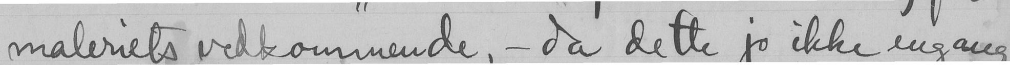maleriets vedkommende, - da dette jo ikke engang
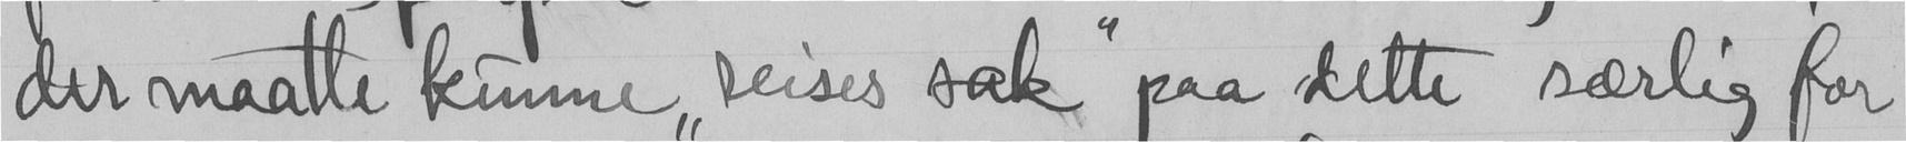der maatte kunne "reises sak" paa dette særlig for
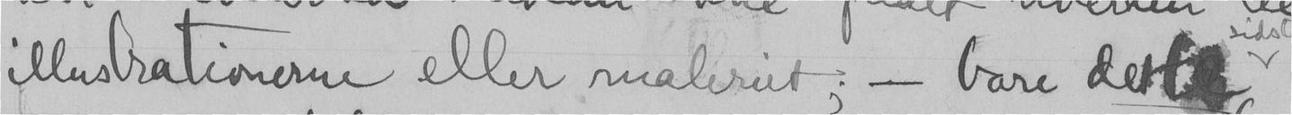illustrationerne eller maleriet, - bare dette
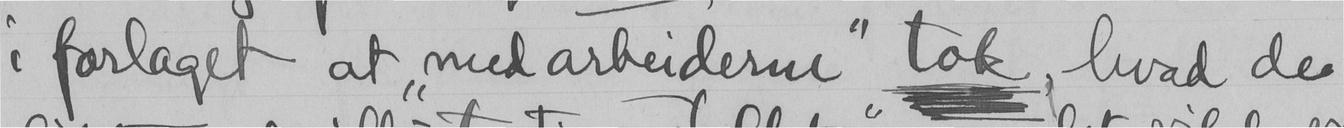i forlaget at "medarbeiderne" tok, hvad de
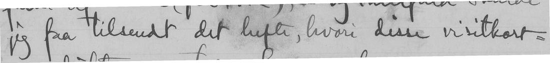jeg faa tilsendt det hefte, hvori disse visitkort-
